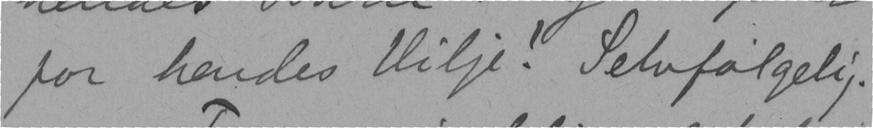for hendes Vilje! Selvfølgelig.
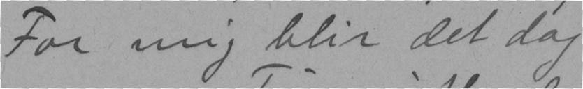For mig blir det dog
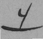4
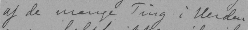af de mange Ting i verden
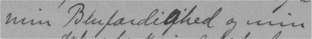min Blufærdighed og min
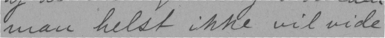man helst ikke vil vide
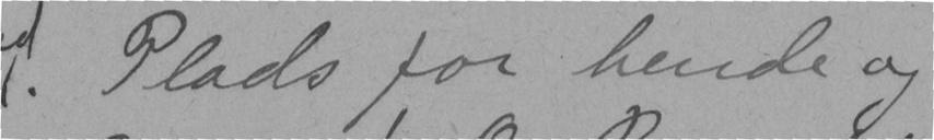. Plads for hende og
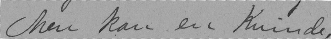Men kan en Kvinde
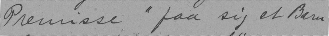Premisse "faa sig et Barn
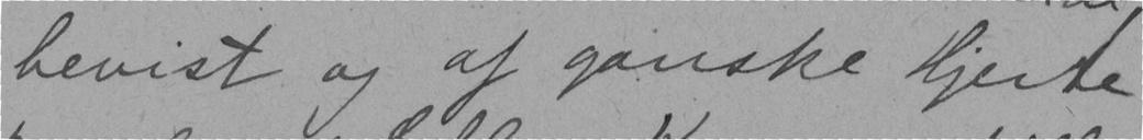bevist og af ganske Hjerte
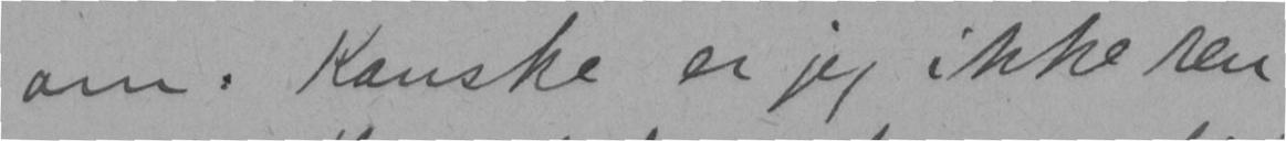om. Kanske er jeg ikke ren
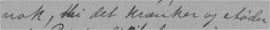nok, thi det krænker og støder
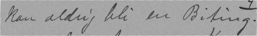kan aldrig bli en Biting.
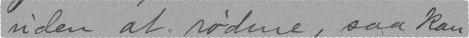uden at rødme, saa kan
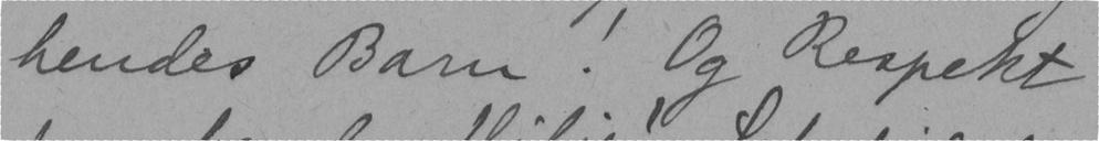hendes Barn! Og Respekt
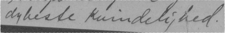dybeste Kvindelighed.
